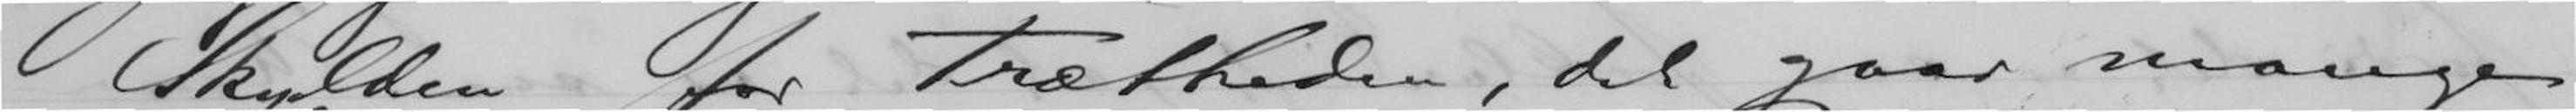Skylden for Trætheden, det gaar mange
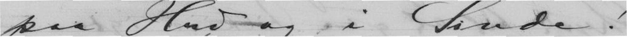paa Hud og i Sinde!
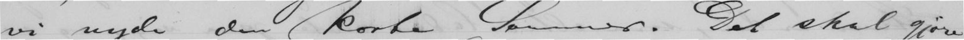vi nyde den korte Sommer. Det skal gjøre
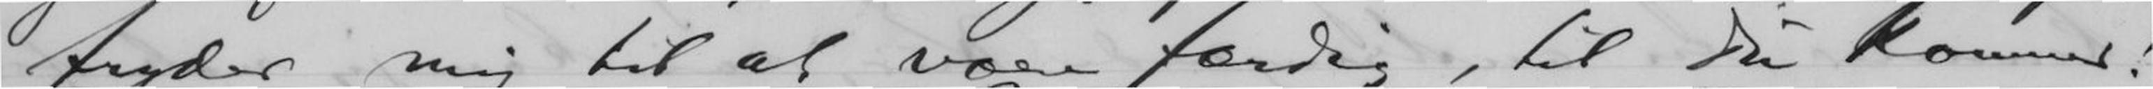fryder mig til at være færdig, til Du kommer!
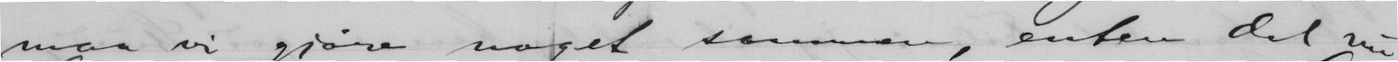maa vi gjøre noget sammen, enten det nu
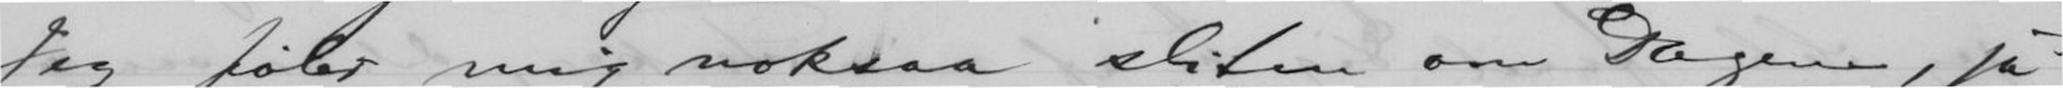Jeg føler mig noksaa sliten om Dagene, ja-
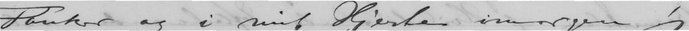Tanker og i mit Hjerte imorgen!
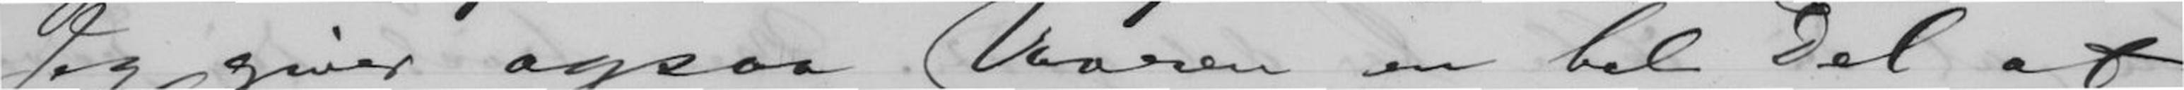Jeg giver ogsaa Vaaren en hel Del af
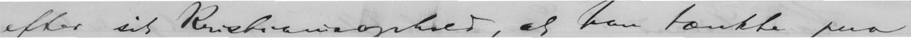efter sit Kristianiaophold, at han tænkte paa
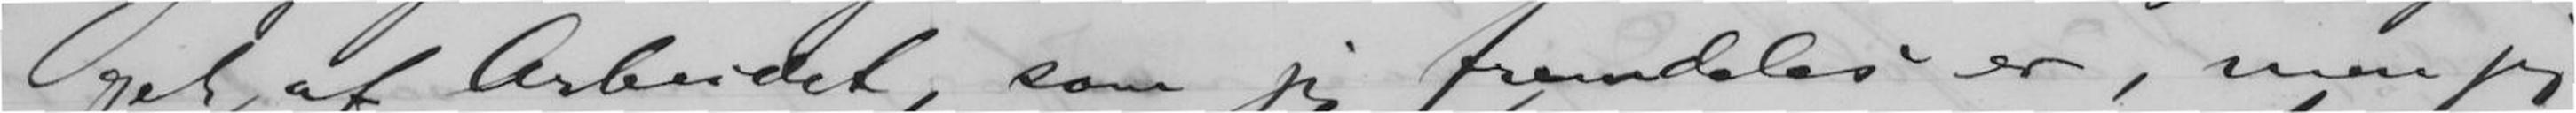get af Arbeidet, som jeg fremdeles er, men jeg
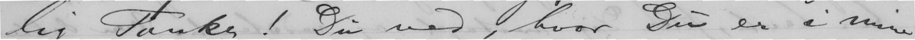lig Tanke! Du ved, hvor Du er i mine
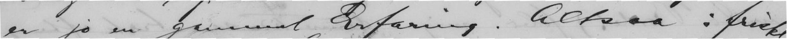er jo en gammel Erfaring. Altsaa: friskt
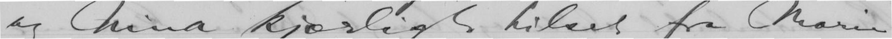og Nina kjærligt hilset fra Marie
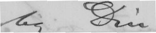og Din
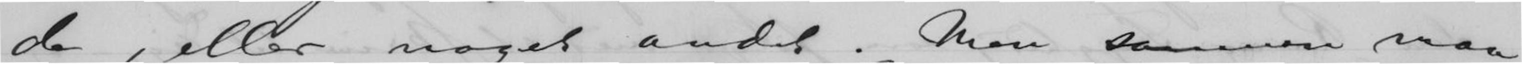de, eller noget andet. Men sammen maa
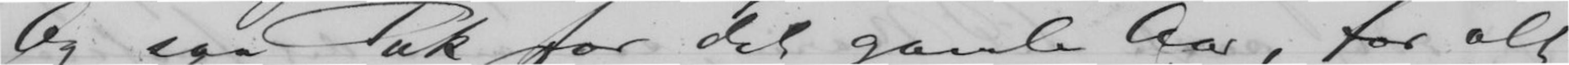Og saa Tak for det gamle Aar, for alt
